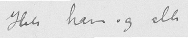Hils ham og alle
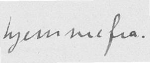hjemmefra.
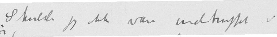Skulde jeg ikke være indtruffet i
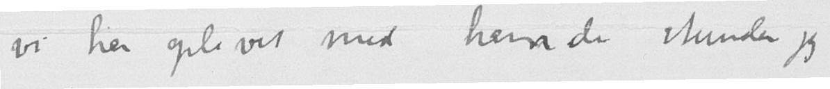vi har oplevet med ham de stunder jeg
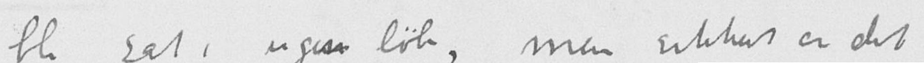bli sat i ugens løb, men sikkert er det
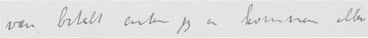være betalt enten jeg er kommen eller
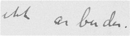ikke arbeider.
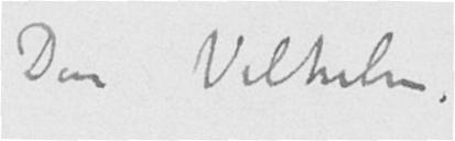Din Vilhelm.
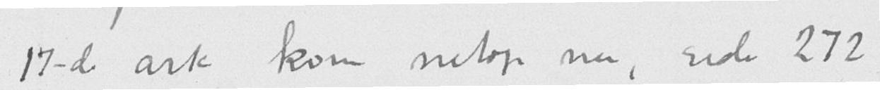17-de ark kom netop nu, side 272.
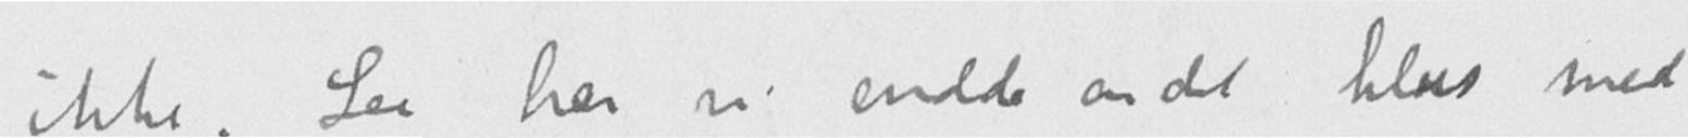ikke. Saa har vi endda endel klus med
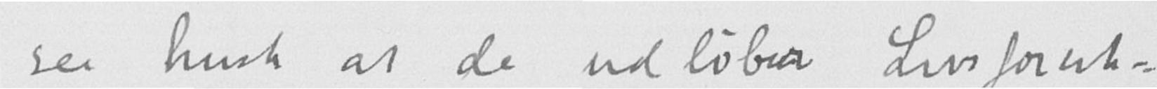saa husk at da udløber Livsforsik-
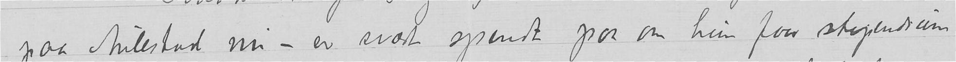paa Aulestad nu – er svært spendt paa om hun faar stependiem
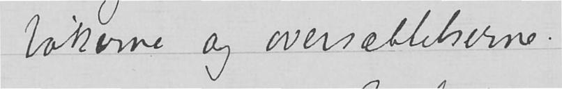bøkerne og oversættelserne.
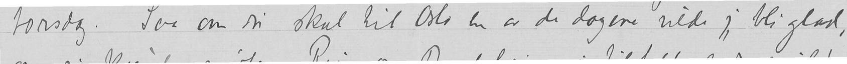torsdag. Saa om du skal til Oslo en av de dagene vilde jeg bli glad,
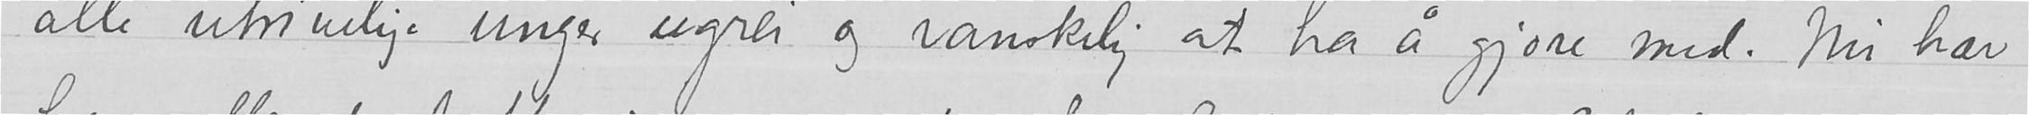alle utrivelige unger ugrei og vanskelig at ha å gjøre med. Nu har
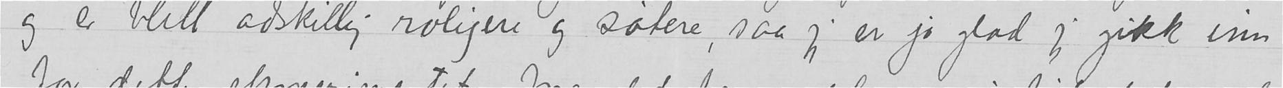og er blitt adskillig roligere og søtere, saa jeg er jo glad jeg gikk inn
